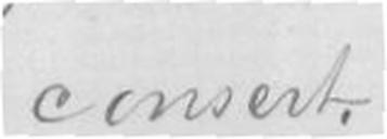consert.
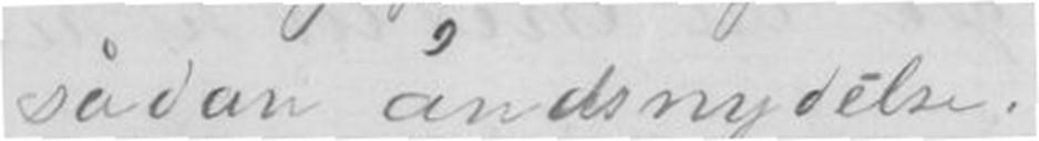sådan åndsnydelse.
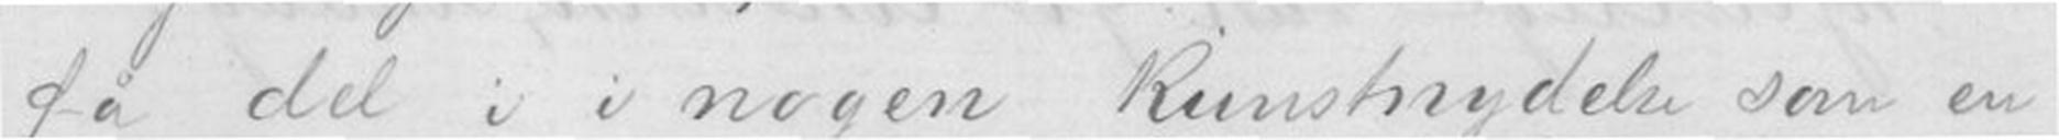få del i i nogen kunstnydelse som en
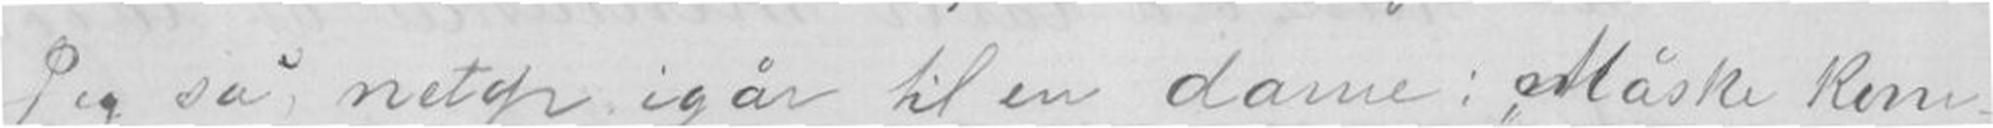Jeg så netop igår til en dame: Måske kom-
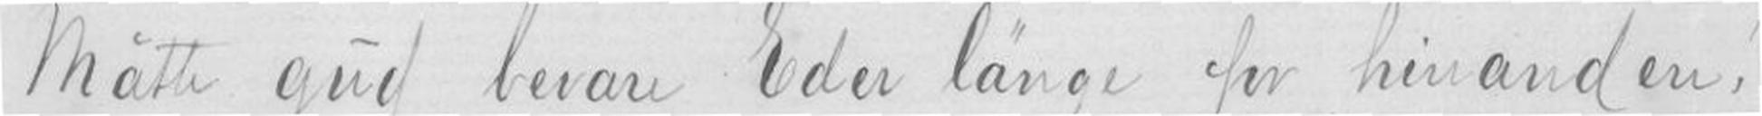Måtte gud bevare Eder länge for hinanden!
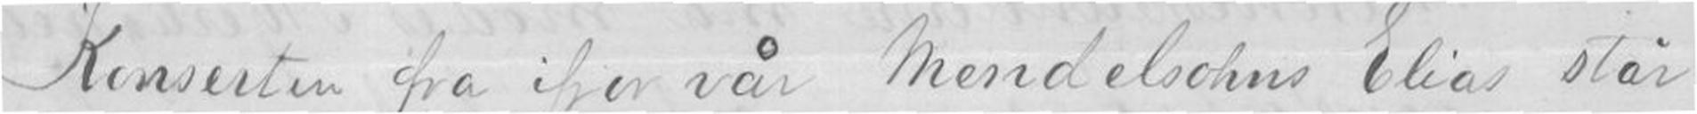Konserten fra ifjor vår Mendelsohns Elias står
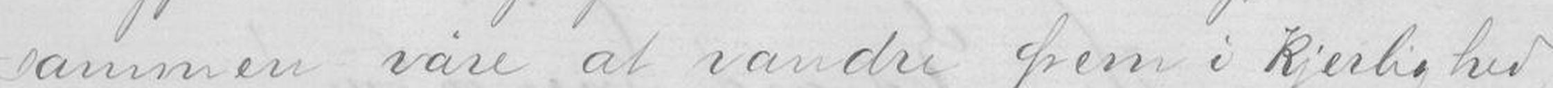sammen väre at vandre frem i kjerlighed
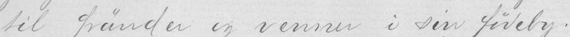til fränder og venner i sin fødeby.
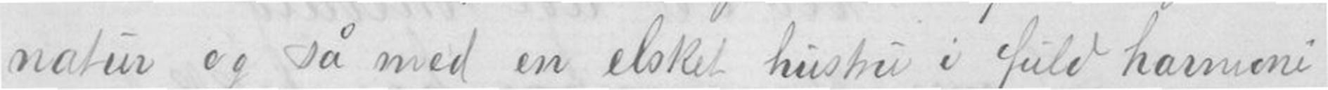natur og så med en elsket hustru i fuld harmoni
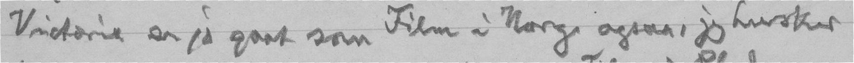Victoria er jo gaat som Film i Norge ogsaa, jeg husker
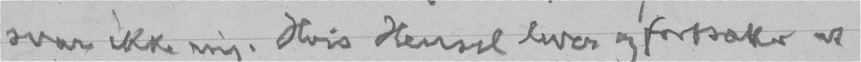svar ikke mig. Hvis Hensel lever og fortsætter at
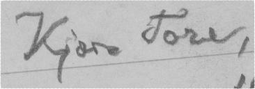Kjære Tore,
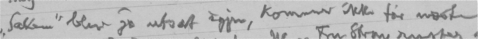"Saken" blev jo utsat igjen, kommer ikke før næste
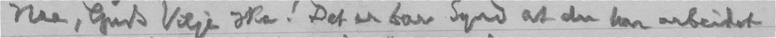Naa, Guds Vilje ske! Det er bare Synd at du har arbeidet
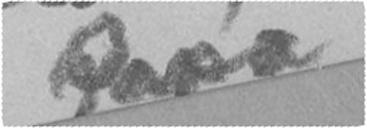Papa
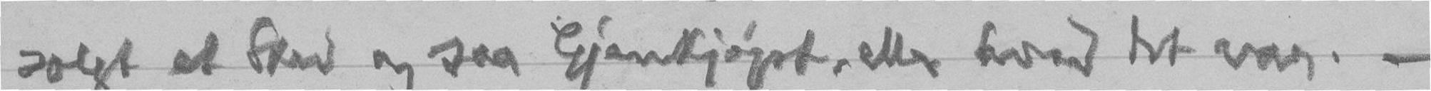solgt et Sted og saa Gjenkjøpt, eller hvad det var. -
